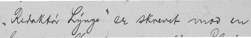"Redaktør Lynge" er skrevet mod en
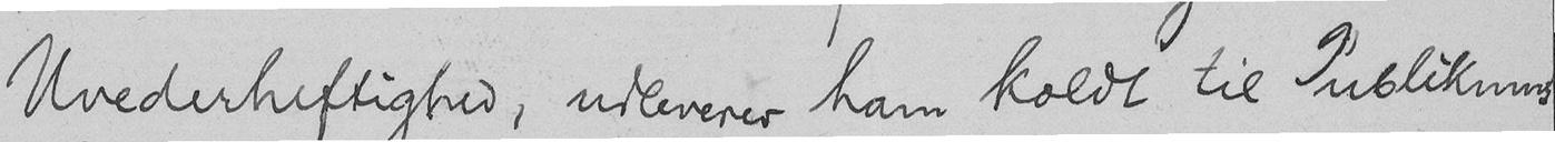Uvederheftighed, udleverer ham koldt til Publikums
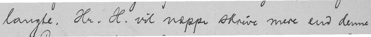langte. Hr. H. vil næppe skrive mere end denne
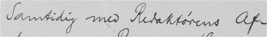Samtidig med Redaktørens Af-
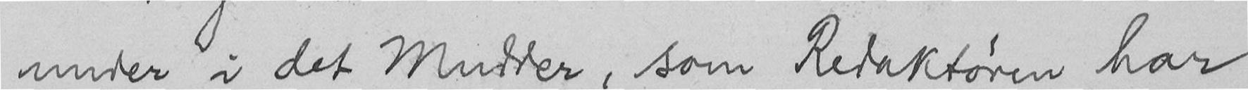under i det Mudder, som Redaktøren har
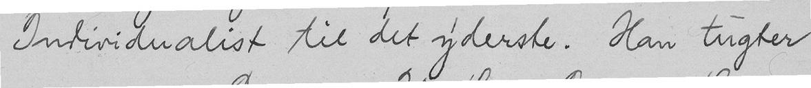Individualist til det yderste. Han tugter
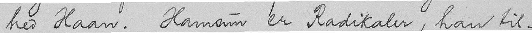hed Haan. Hamsun er Radikaler, han til-
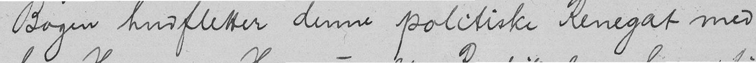Bogen hudfletter denne politiske Renegat med
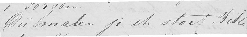Du maler jo et stort Bille-
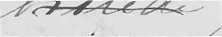billede
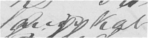landskab
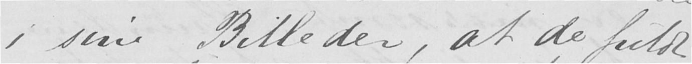i sine Billeder, at de fuldt
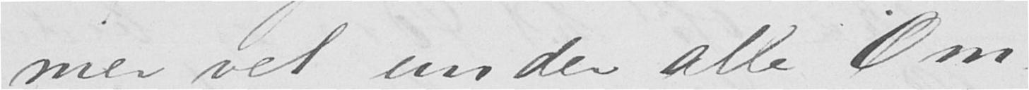mer vel under alle Om-
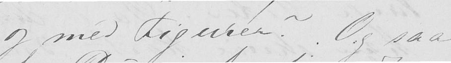og med Figurer? Og saa
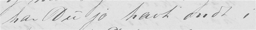har Du jo havt ondt i
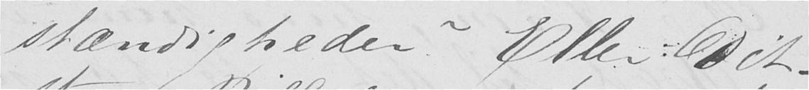stændigheder? Eller Dit
